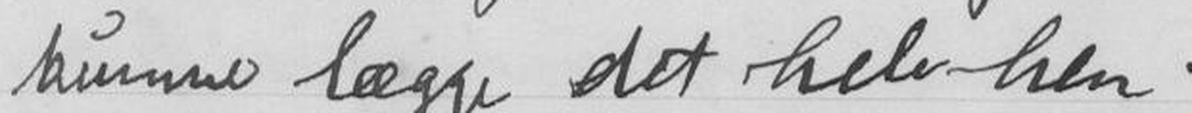kunne lægge det hele hen.
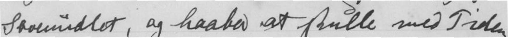Sovemidlet, og haaber at skulle med Tiden
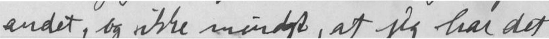andet, og ikke mindst, at jeg har det
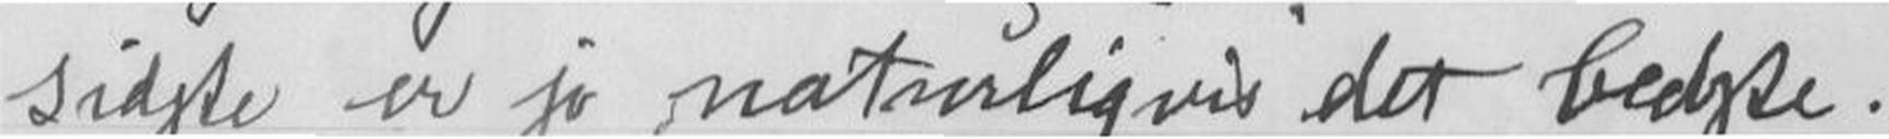Sidste er jo naturligvis det bedste.
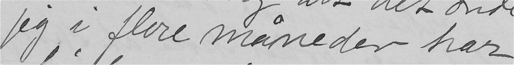jeg i flere måneder har
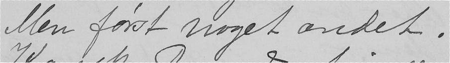Men først noget andet.
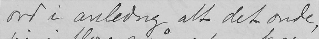ord i anledning alt det onde,
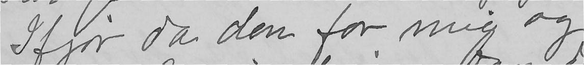Ifjor da den for mig og
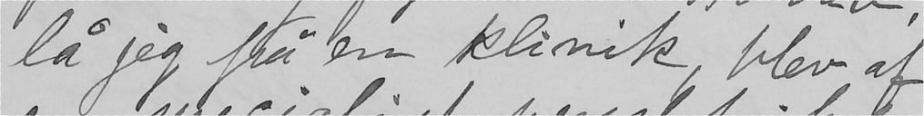lå jeg på en klinik, blev af
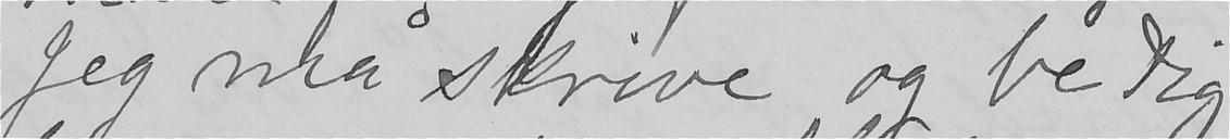Jeg må skrive, og be dig
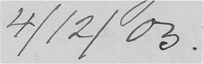4/12/03.
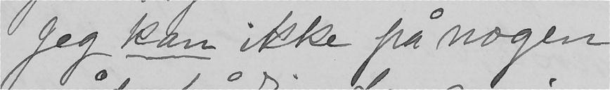Jeg kan ikke på nogen
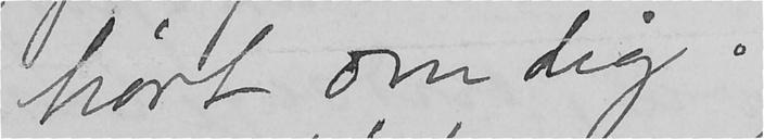hørt om dig.
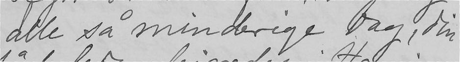alle så minderige dag, din
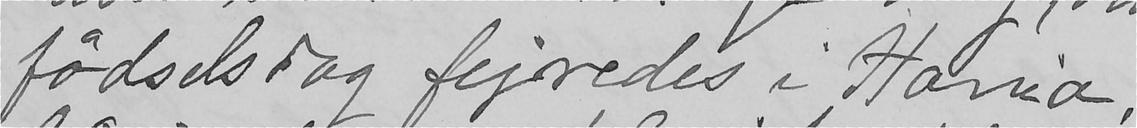fødselsdag fejredes i Xania,
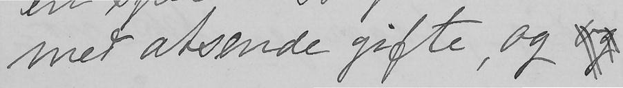med ætsende gifte, og og
